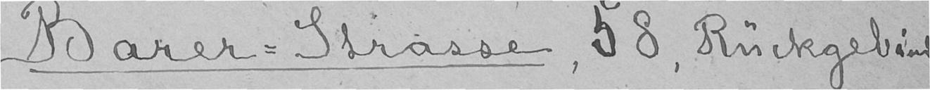Barer-Strasse, 58, Rückgebäude
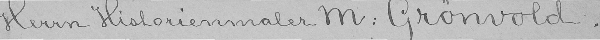Herrn Historienmaler M: Grønvold.
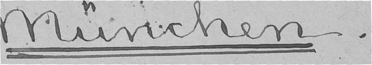München.
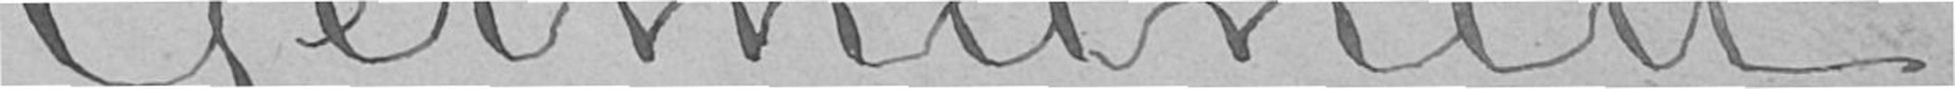Germania.
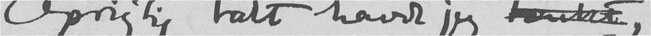Oprigtig talt havde jeg tænkt,
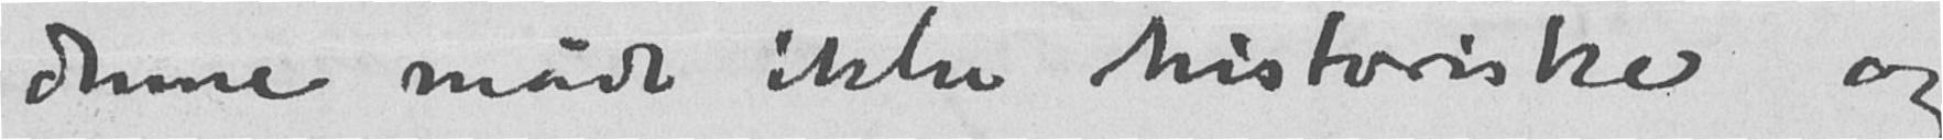denne måde ikke historiske og
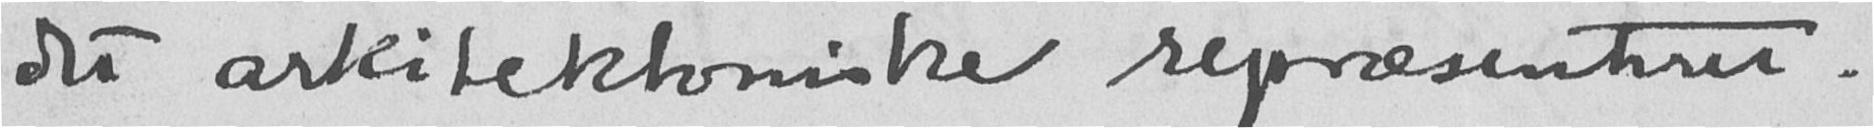det arkitektoniske repræsenteret.
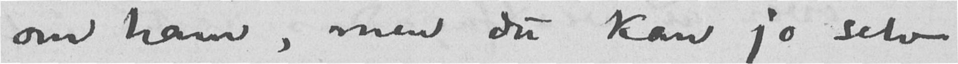om ham, men du kan jo selv
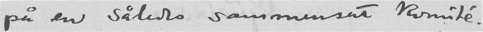på en således sammensat komité.
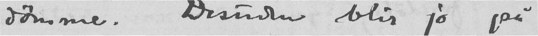dømme. Desuden blir jo på
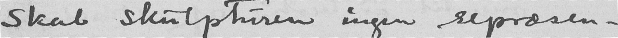Skal skulpturen ingen repræsen-
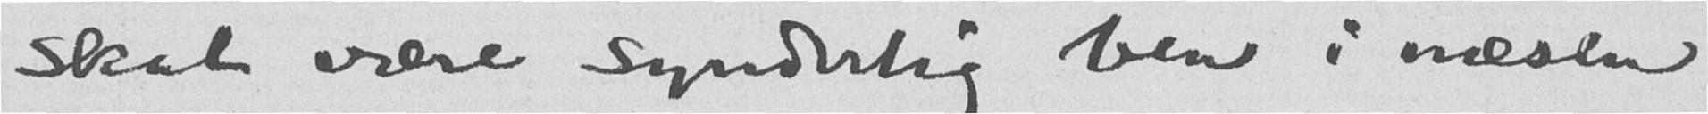skal være synderlig ben i næsen
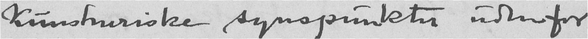kunstneriske synspunkter udenfor
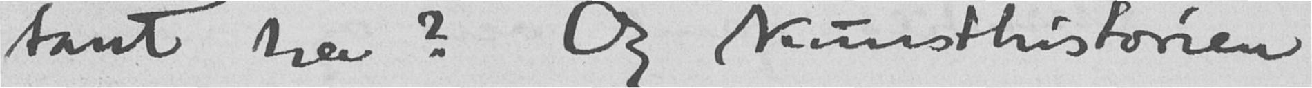tant ha? Og kunsthistorien
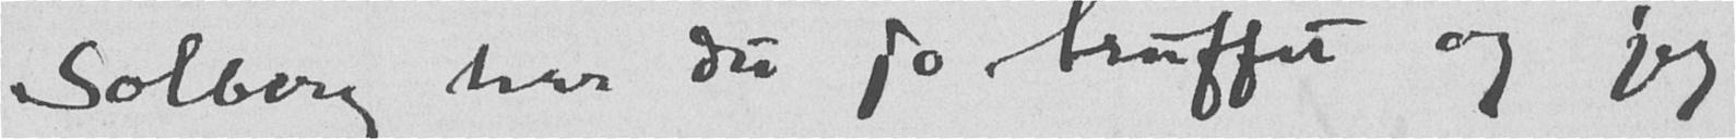Solberg har du jo truffet og jeg
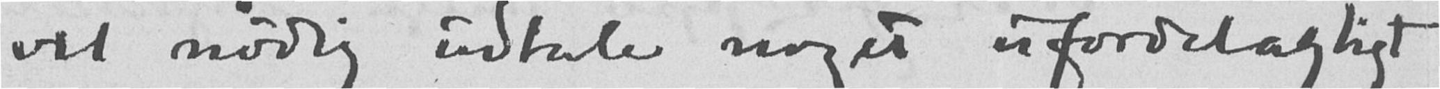vil nødig udtale noget ufordelagtigt
